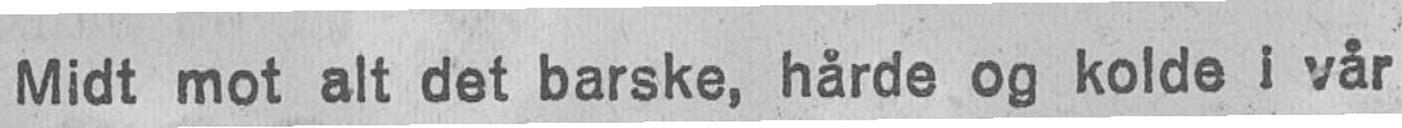Midt mot alt det barske, hårde og kolde i vår
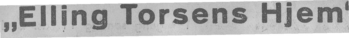"Elling Torsens Hjem"
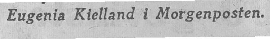Eugenia Kielland i Morgenposten.
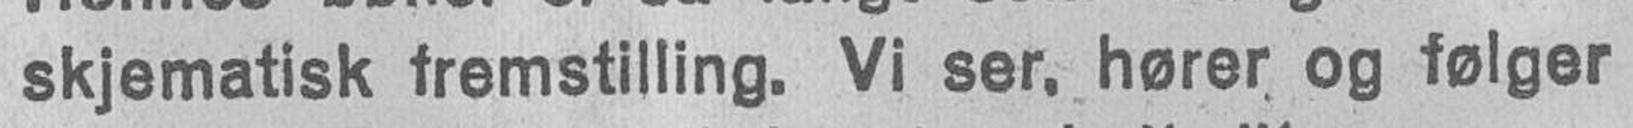skjematisk fremstilling. Vi ser, hører og følger
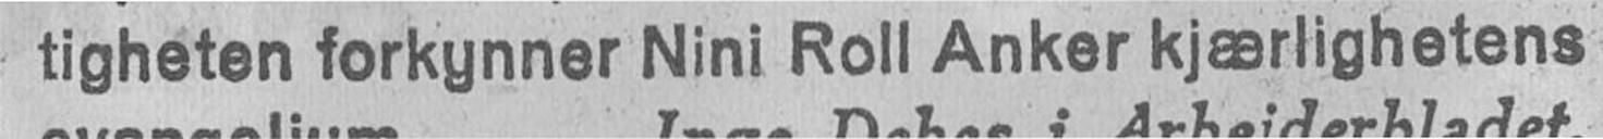tigheten forkynner Nini Roll Anker kjærlighetens
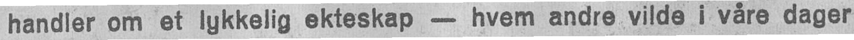handler om et lykkelig ekteskap — hvem andre vilde i våre dager
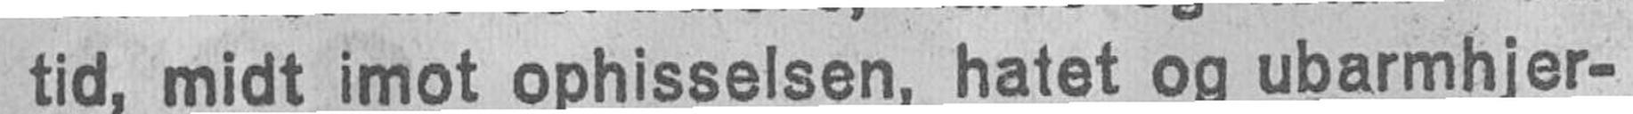tid, midt imot ophisselsen, hatet og ubarmhjer-
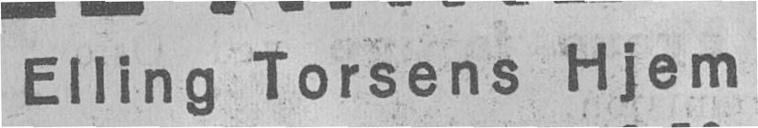Elling Torsens Hjem
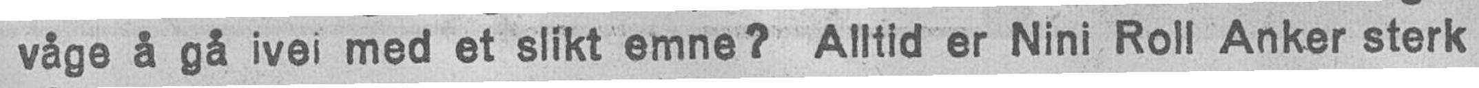våge å gå ivei med et slikt emne? Alltid er Nini Roll Anker sterk
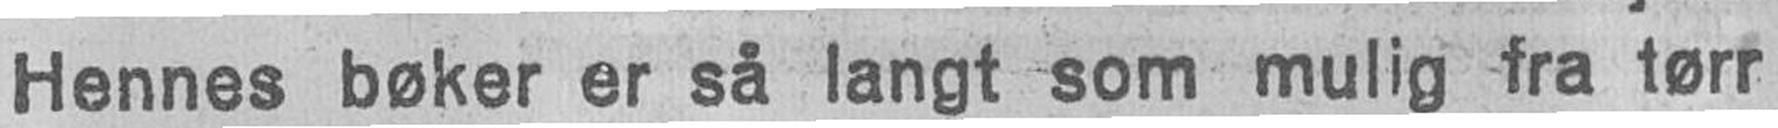Hennes bøker er så langt som mulig fra tørr
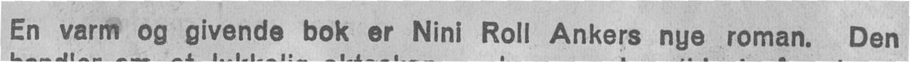En varm og givende bok er Nini Roll Ankers nye roman. Den
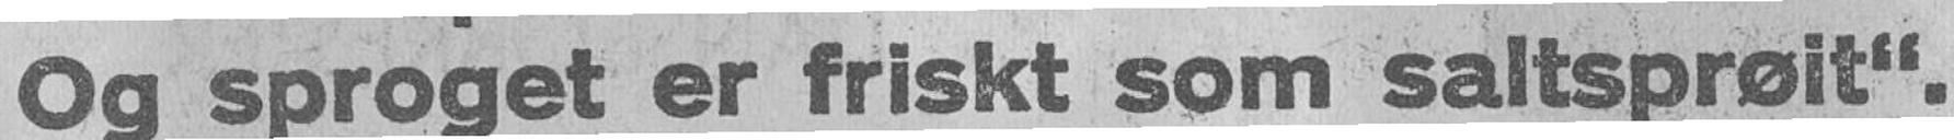Og sproget er friskt som saltsprøit".
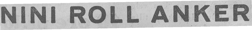NINI ROLL ANKER
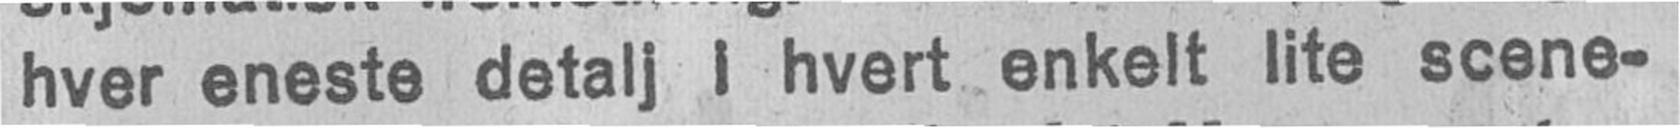hver eneste detalj i hvert enkelt lite scene-
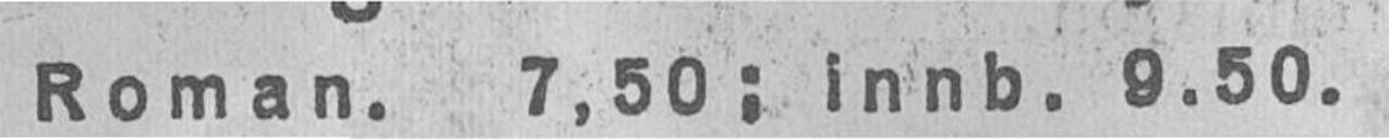Roman. 7,50; innb. 9.50.
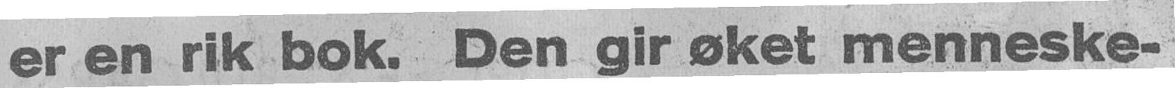er en rik bok. Den gir øket menneske-
